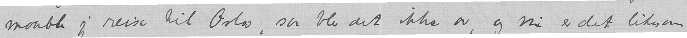maatte jeg reise til Oslo, saa blev det ikke av, og nu er det likesom
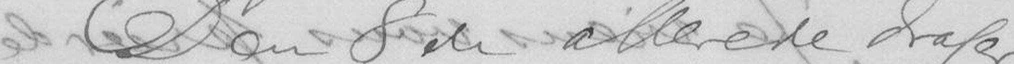Den 8 de allerde drager
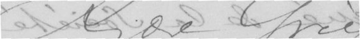Kjære Grieg.
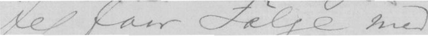Jeg faar Følge med
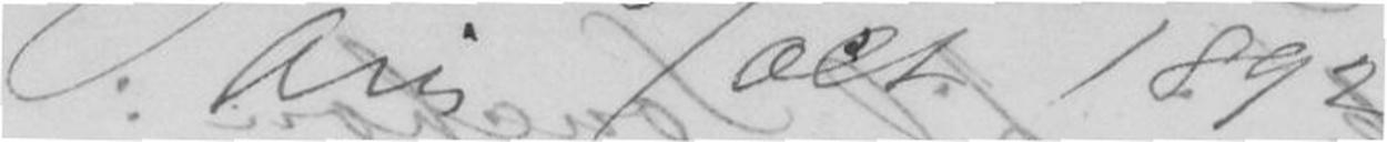Paris 5/oct 1892
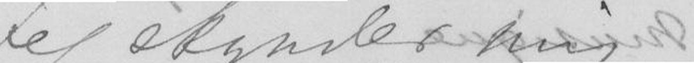Jeg skynder mig
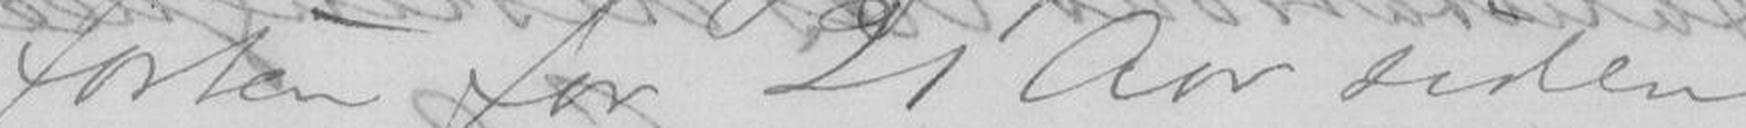farten for 21 Aar siden.
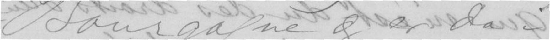Bourgagne og er da i
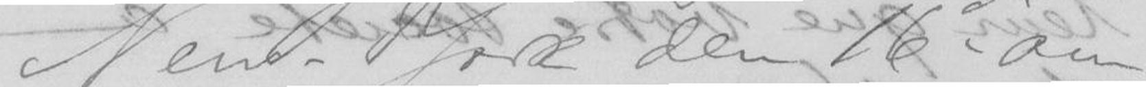New-York den 16de om
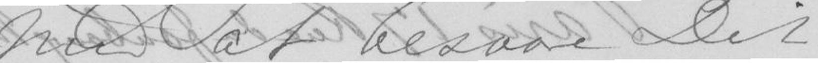med at besvare Dit
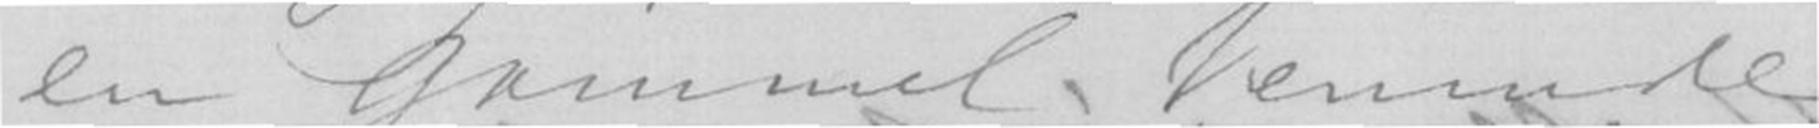en gammel Veninde
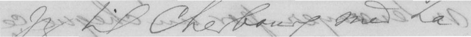jeg til Cherbourg med La
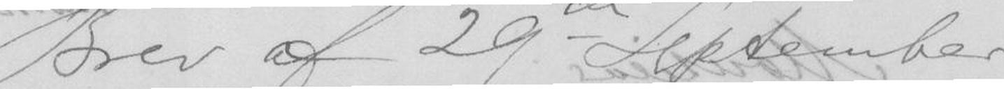Brev af 29de September.
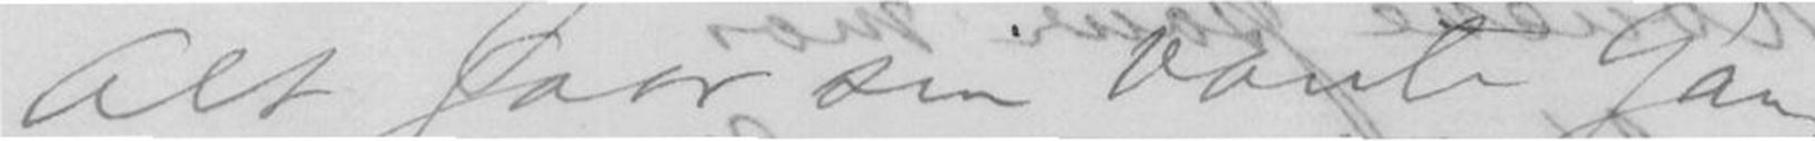Alt gaar sin vante Gang.
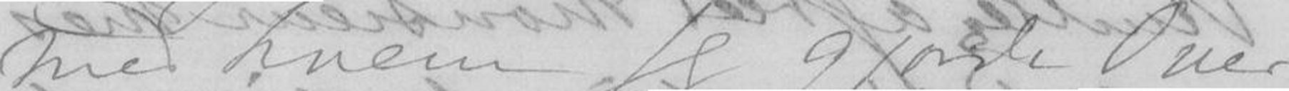med hvem jeg gjorde Over-
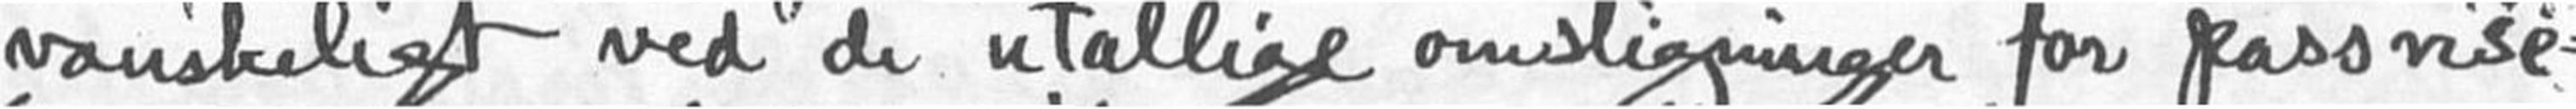vanskeligt ved de utallige omstigninger for passvise-
